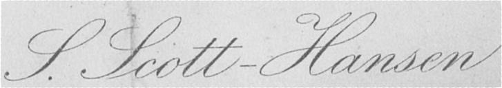S. Scott-Hansen
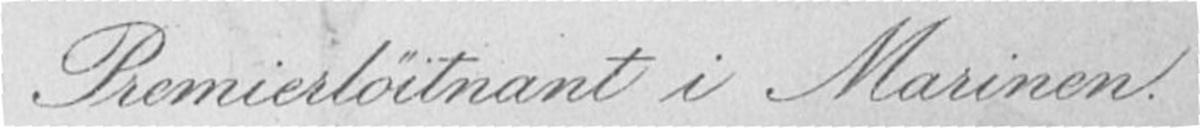Premierløitnant i Marinen.
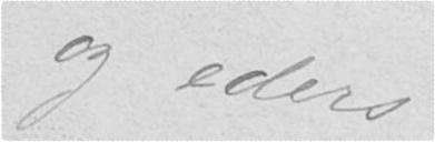og eders
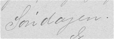Søndagen.
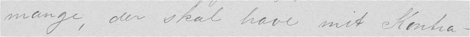mange, der skal have mit Kontra-
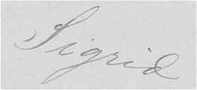Sigrid
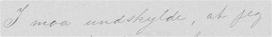I maa undskylde, at jeg
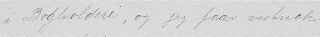i Bogholdere, og jeg faar vistnok
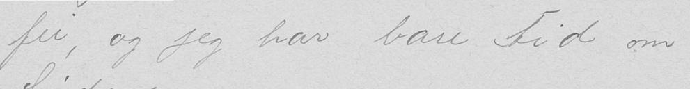fei, og jeg har bare tid om
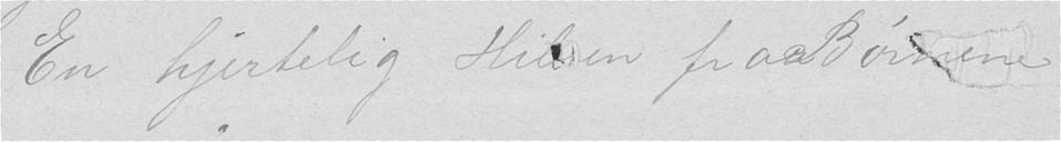En hjertelig Hilsen fra Børnene
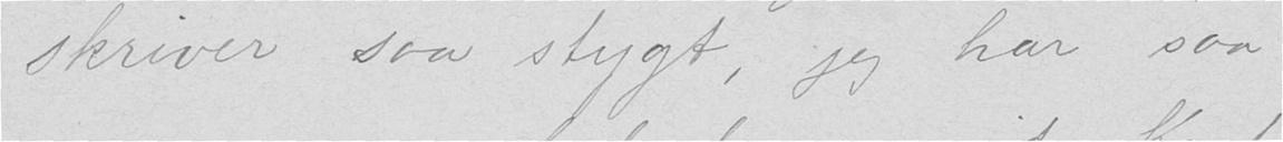skriver saa stygt, jeg har saa
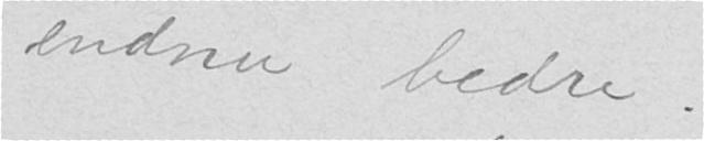endnu bedre.
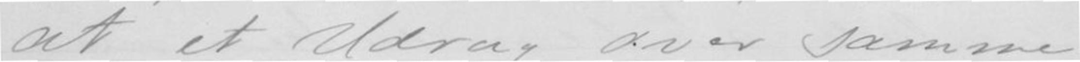at et Udrag over samme
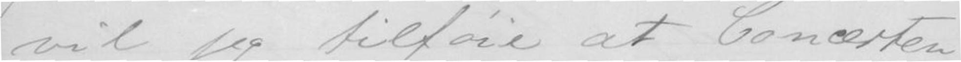vil jeg tilføie at Concerten
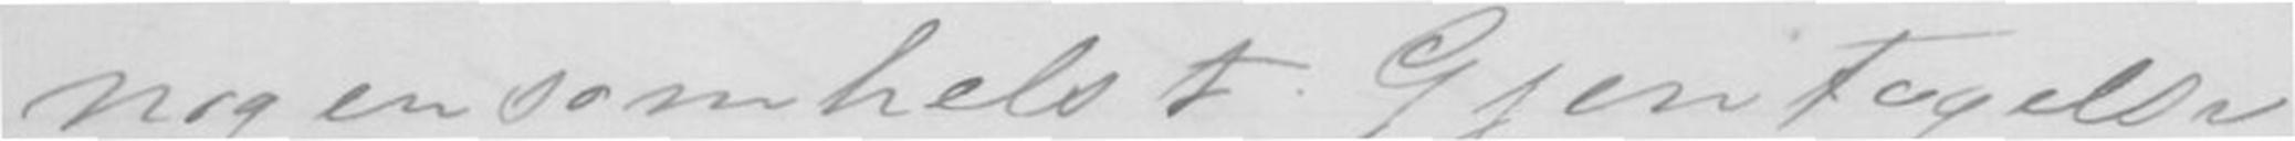nogensomhelst Gjentagelse
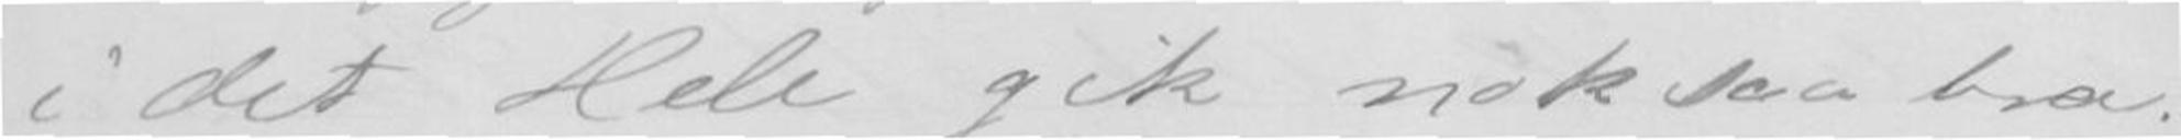i det Hele gik noksaa bra.
